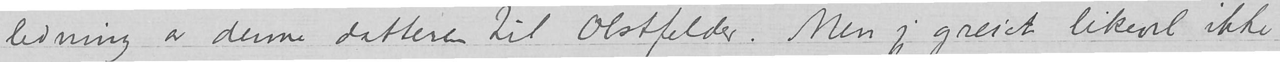ledning av denne datteren til Obstfelder. Men jeg greiet likevel ikke
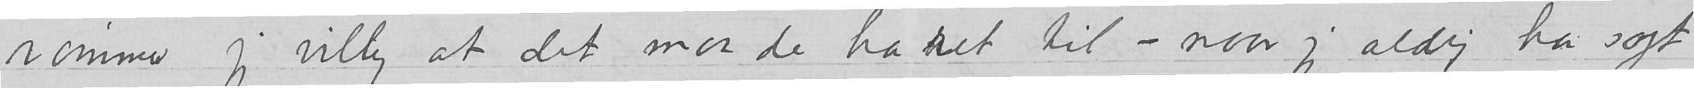rømmer jeg villig at det maa de ha ret til - naar jeg aldrig har sagt
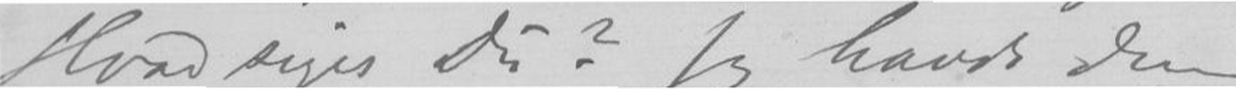Hvad siger Du? Jeg havde den
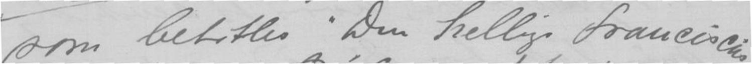som betitles " Den hellige Franciscus
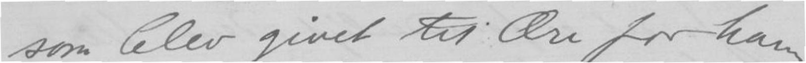som blev givet til Ære for ham
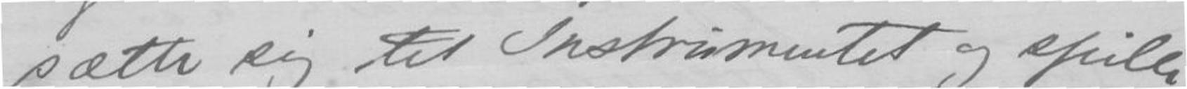sætte sig til Instrumentet og spille
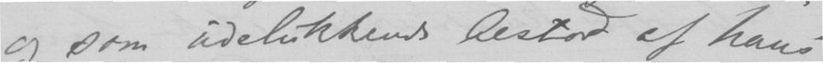og som udelukkende bestod af hans
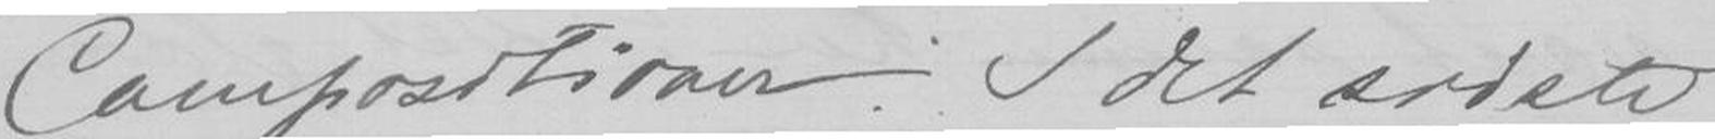Compositioner. I det sidste
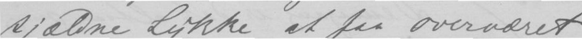sjældne Lykke at faa overværet
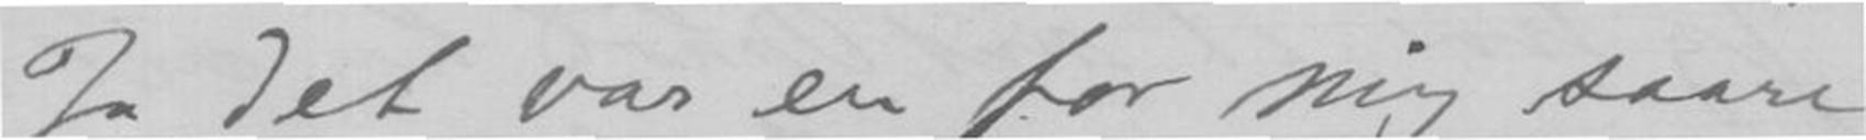Ja det var en for mig saare
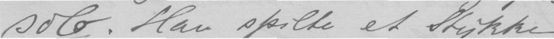solo. Han spilte et Stykke
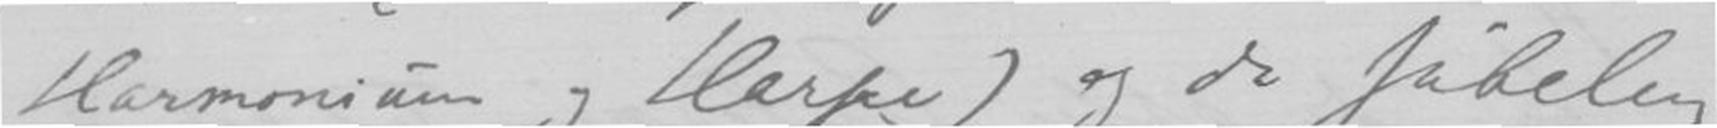Harmonium og Harpe) og da Jubelen
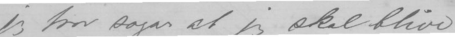jeg tror sogar at jeg skal blive
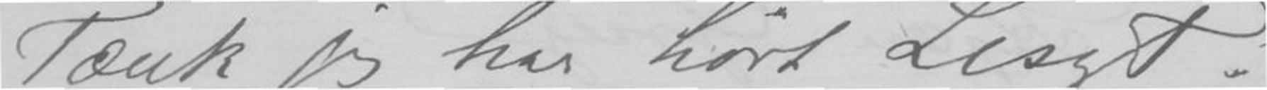Tænk jeg har hørt Liszt!
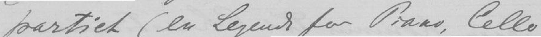partiet (en Legende for Piano, Cello
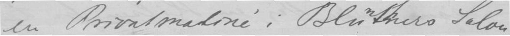en Privatmatiné i Blunthners Salon
